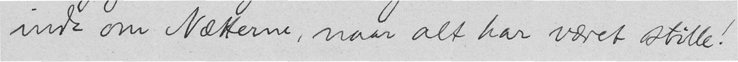inde om Nætterne, naar alt har været stille!
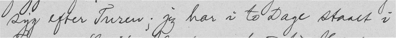syg efter Turen; jeg har i to Dage staaet i
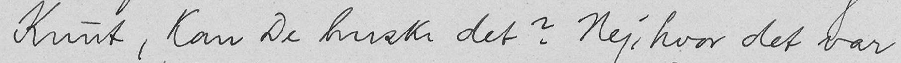Knut, kan De huske det? Nej, hvor det var
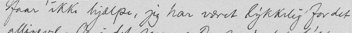faar ikke hjælpe, jeg har været lykkelig for det
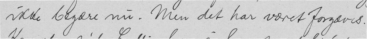ikke begære nu. Men det har været forgæves.
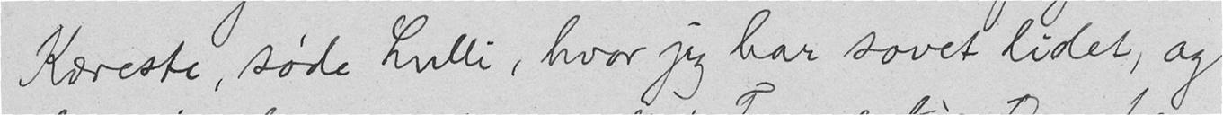Kæreste, søde Lulli, hvor jeg har sovet lidet, og
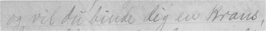og vil du binde dig en krans,
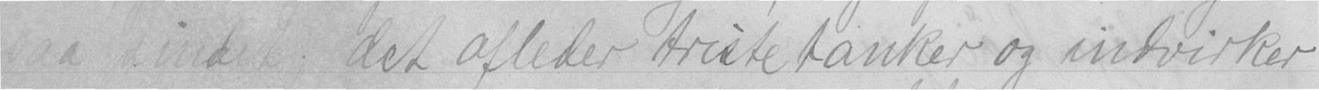paa sindet; det afleder triste tanker og indvirker
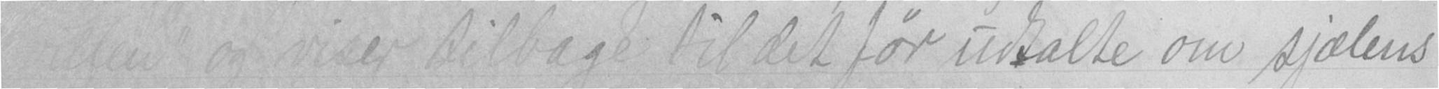en" og viser tilbage til det før udtalte om sjælens
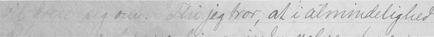vil dreie sig om. Thi jeg tror, at i almindelighed
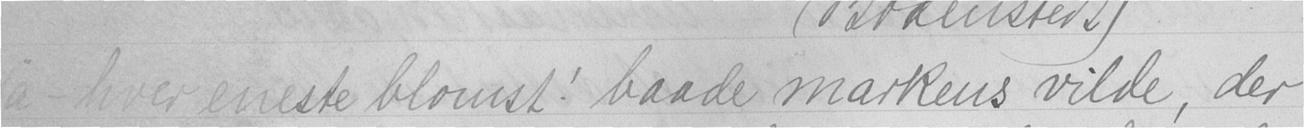Ja - hver eneste blomst! baade markens vilde, der
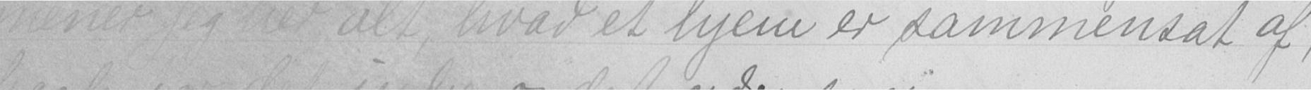mener jeg her alt, hvad et hjem er sammensat af,
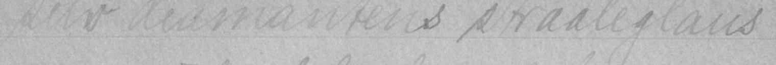selv diamantens straaleglans
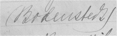(Bodenstedt)
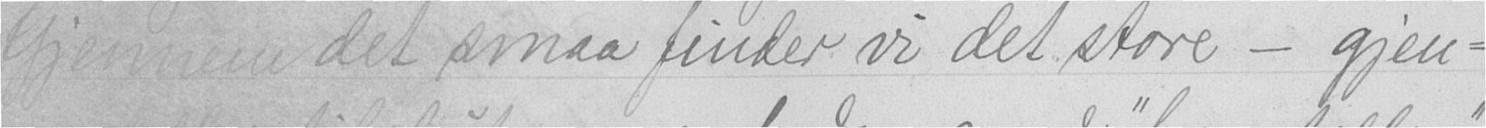Gjennem det smaa finder vi det store - gjen-
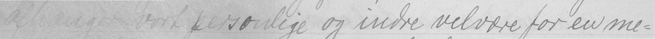afhænger vort personlige og indre velvære for en me-
